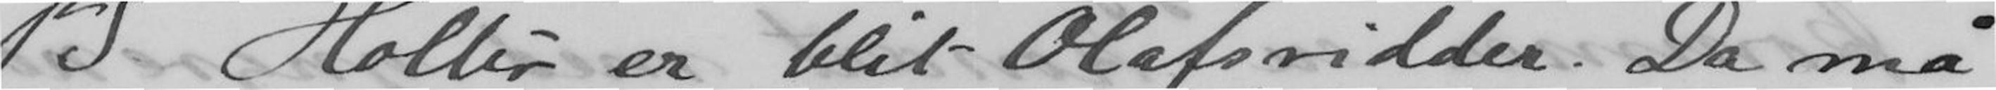P.S.Holter er blit Olafsridder. Da må
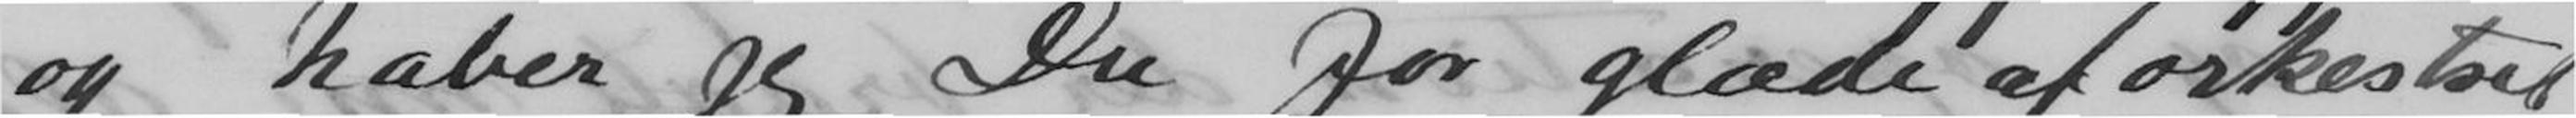og håber jeg Du for glæde af orkestret
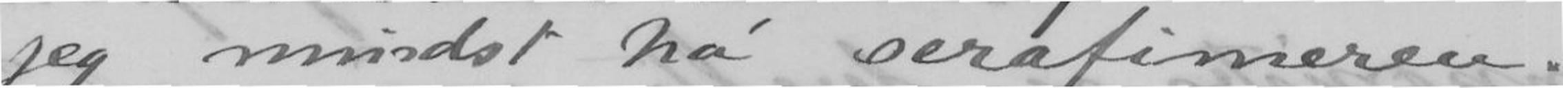jeg mindst ha' serafimeren.
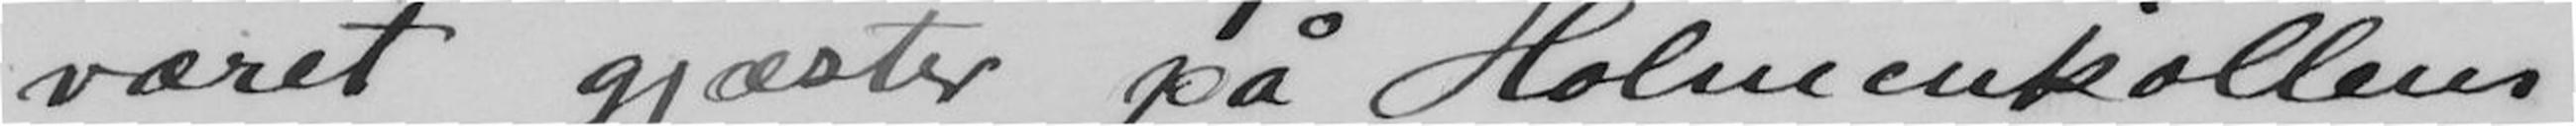været gjæster på Holmenkollens
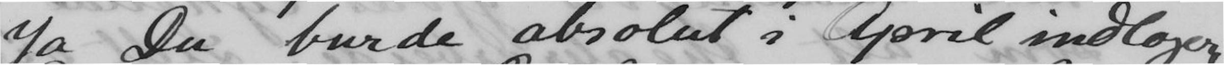Ja, Du burde absolut i April indlogere
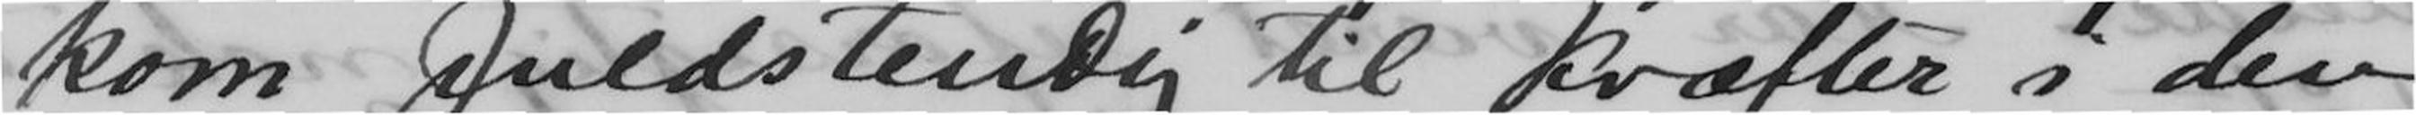kom fuldstendig til kræfter i den
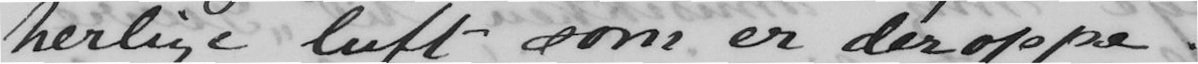herlige luft som er deroppe.
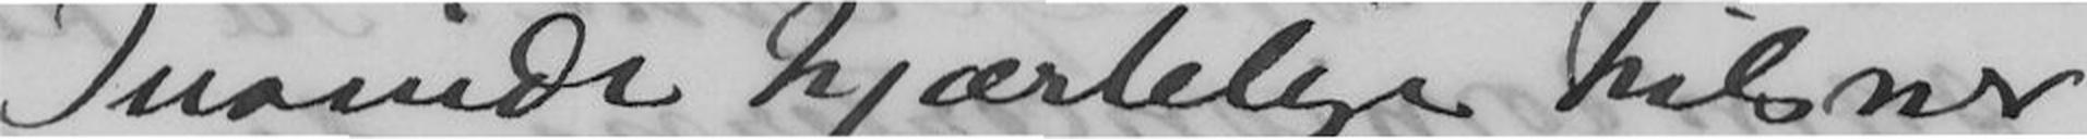Tusinde hjærtelige hilsner
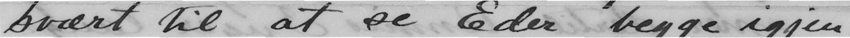svært til at se Eder begge igjen
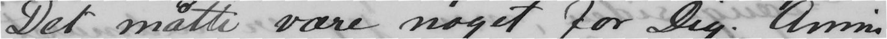Det måtte være noget for Dig. Annie
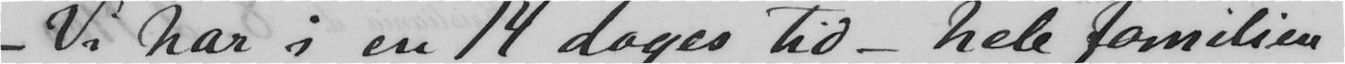- Vi har i en 14 dages tid - hele familien
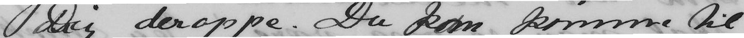Dig deroppe. Du kan komme til
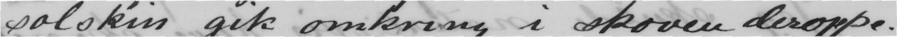solskin gik omkring i skoven deroppe.
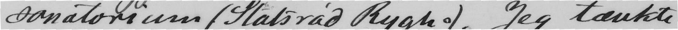sanatorium (Statsråd Rygh.). Jeg tænkte
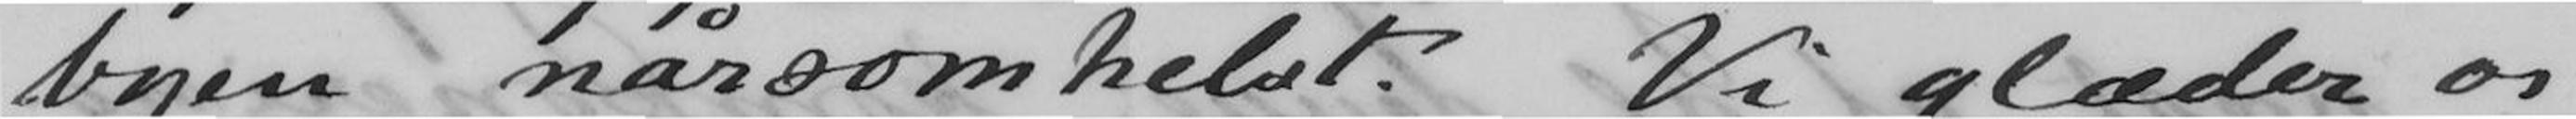byen nårsomhelst. Vi glæder os
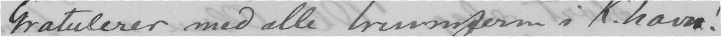Gratulerer med alle triumferne i K.havn!
